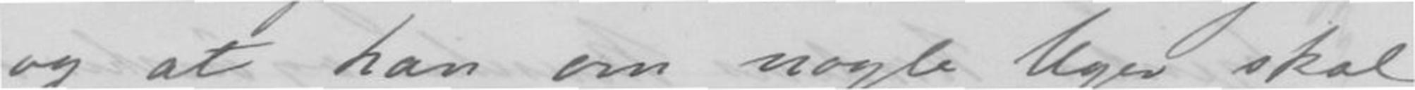og at han om nogle Uger skal
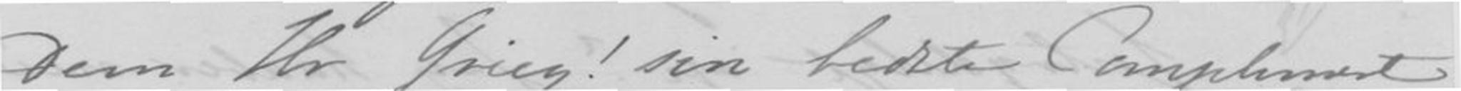Dem Hr Grieg! sin bedste Complement
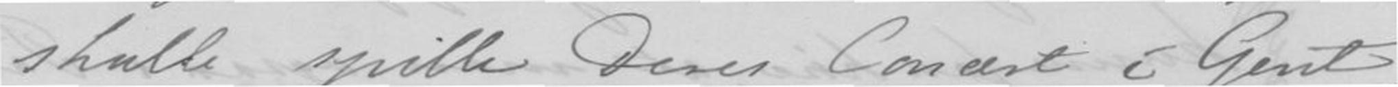skulde spille Deres Concert i Gent,
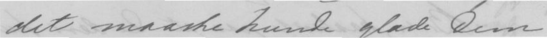det maaske kunde glæde Dem
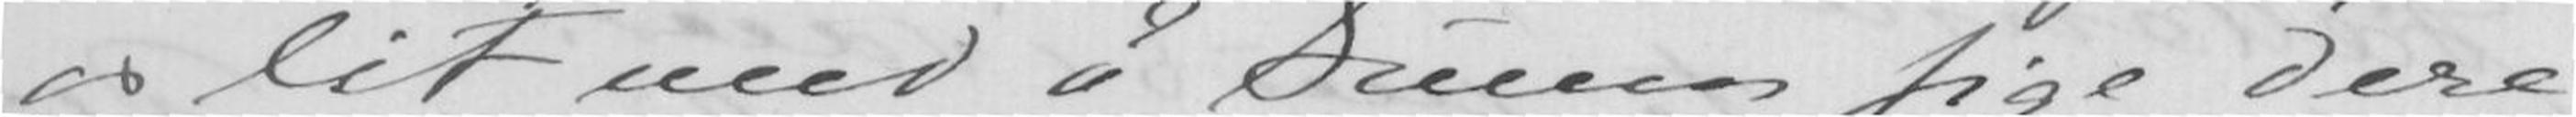os lit med å kunne sige dere
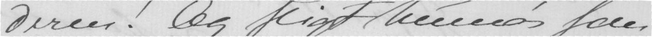deren! Og sligt humør som
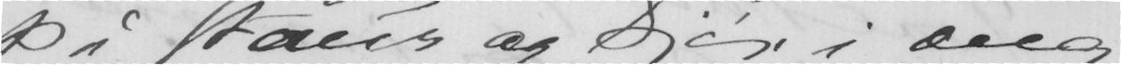på staur og kjør i æng
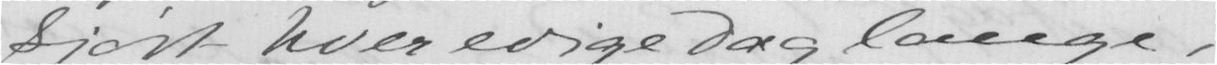kjørt hver evige dag lange,
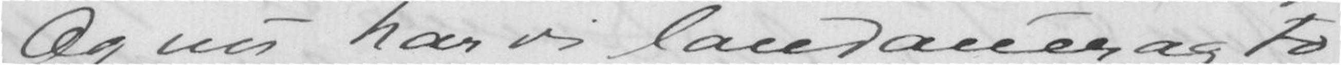Og nu har vi landauer og to
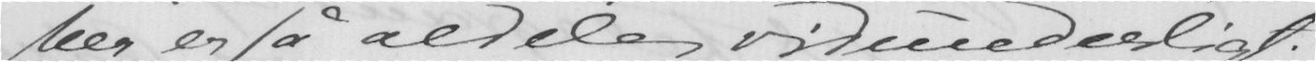her er så aldeles vidunderligt.
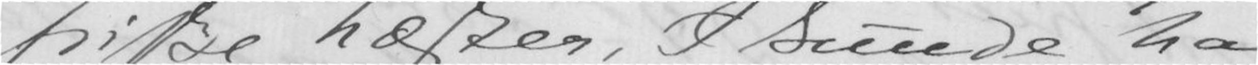friske hæster, I kunde ha
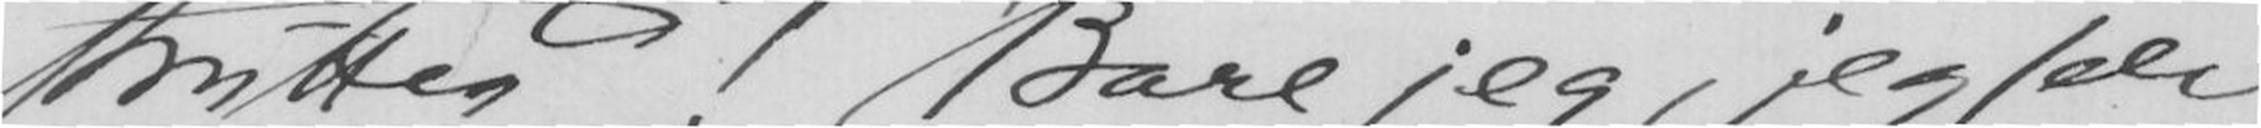strutter! Bare jeg, jeg selv
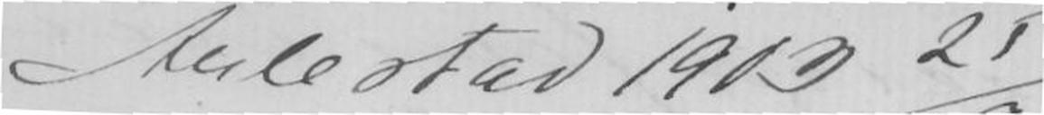Aulestad 1903 25/9
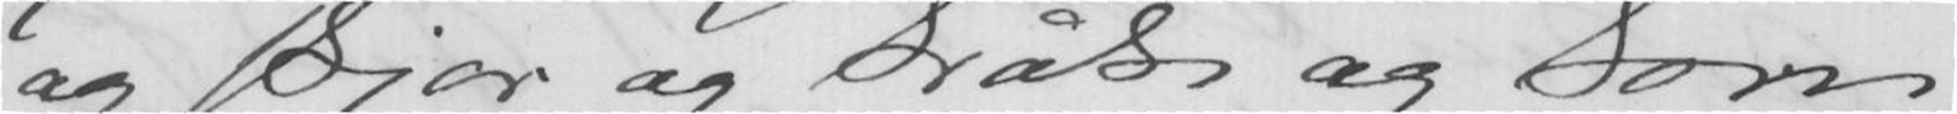og skjor og kråke og korn
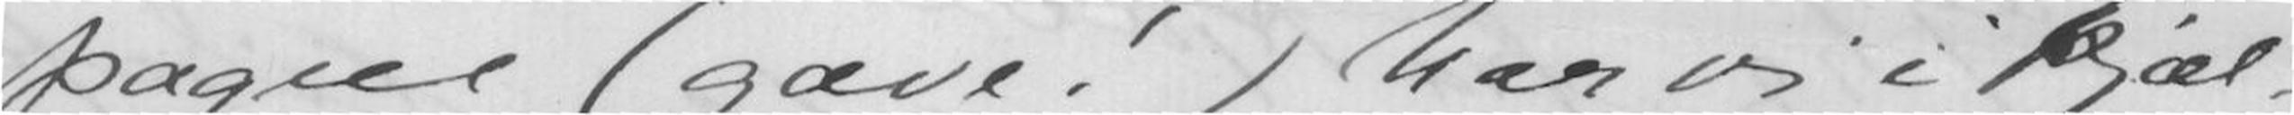pagne (gave!) har vi i kjæl-
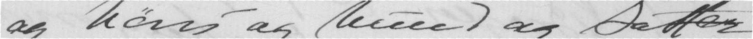og høns og hund og katter
